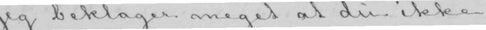Jeg beklager meget at du ikke
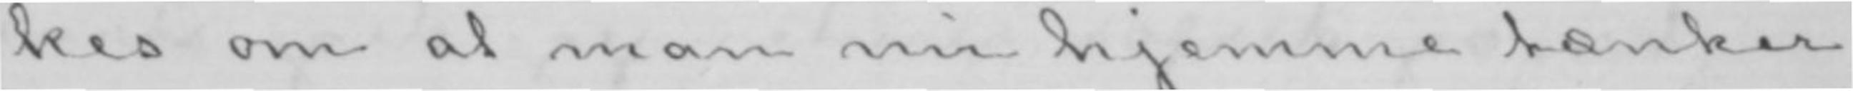kes om at man nu hjemme tænker
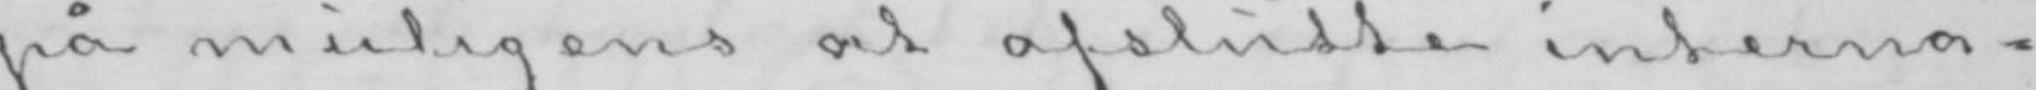på muligens at afslutte interna-
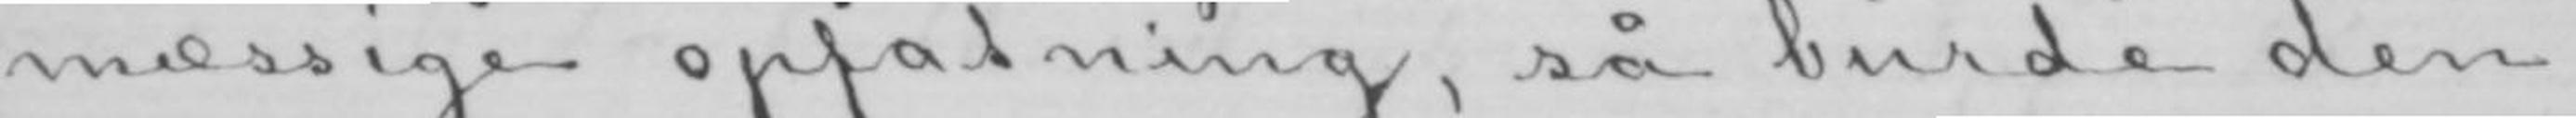mæssige opfatning, så burde den
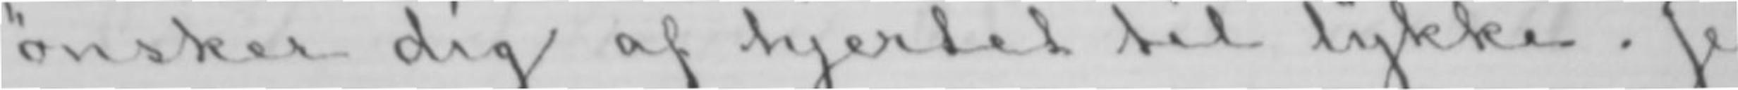ønsker dig af hjertet til lykke. Jeg
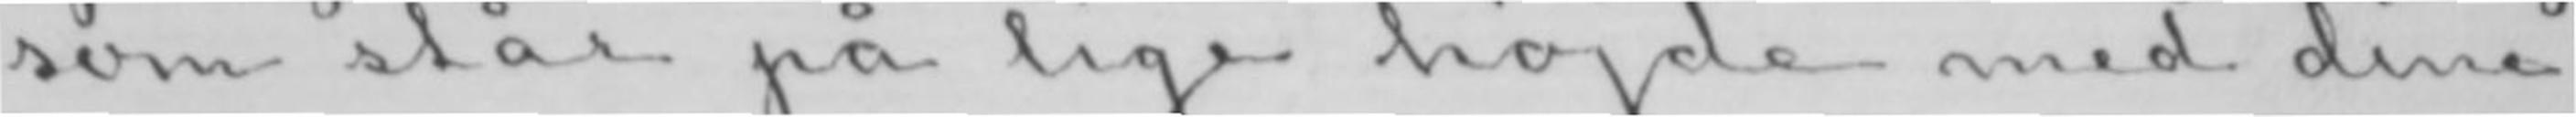som står på lige højde med dine
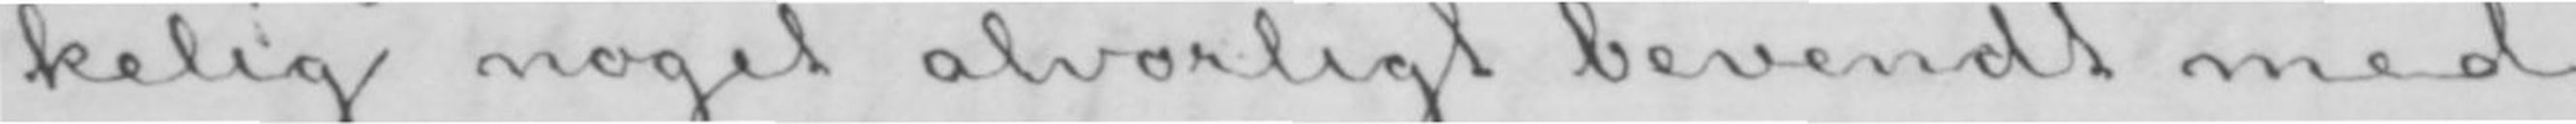kelig noget alvorligt bevendt med
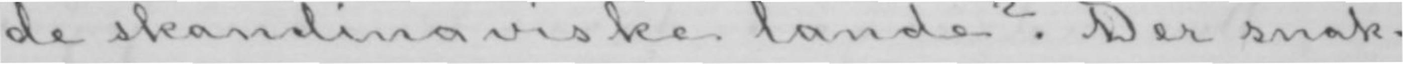de skandinaviske lande? Der snak-
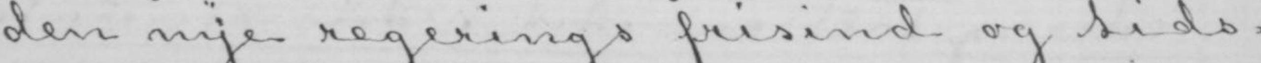den nye regerings frisind og tids-
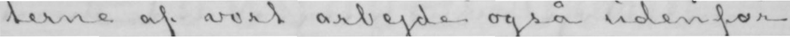terne af vort arbejde også udenfor
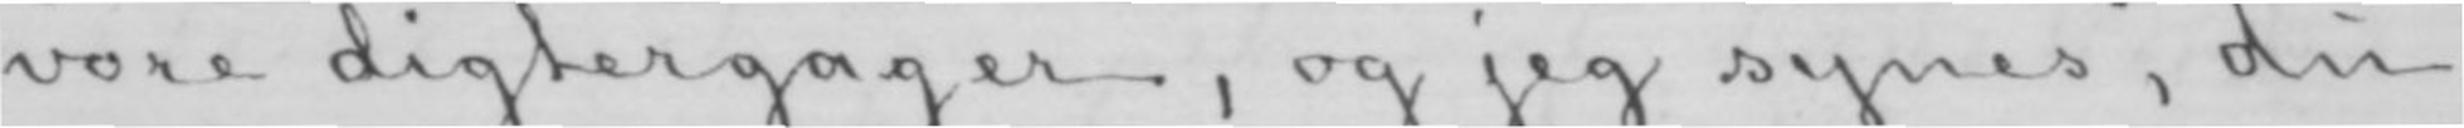vore digtergager, og jeg synes, du
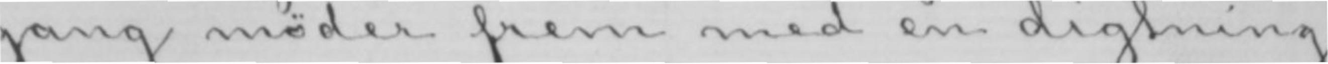gang møder frem med en digtning
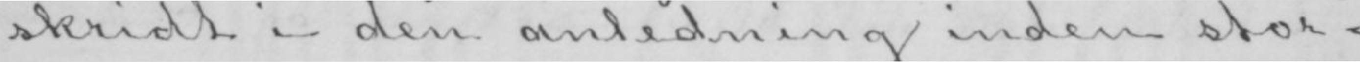skridt i den anledning inden stor-
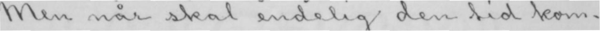Men når skal endelig den tid kom-
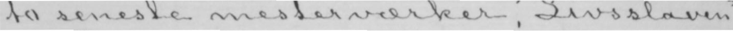to seneste mesterværker, «Livsslaven»
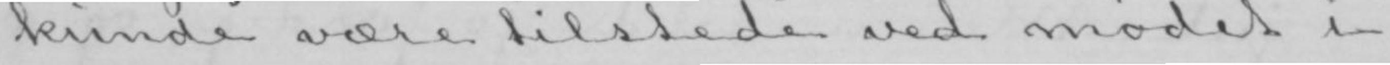kunde være tilstede ved mødet i
